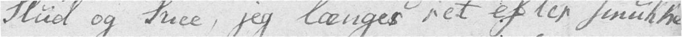Slud og Snee, jeg længes ret efter smukke
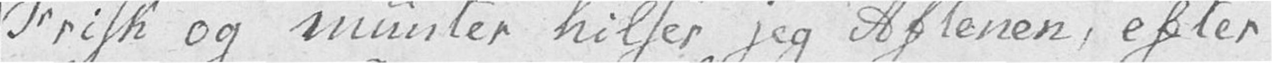Frisk og munter hilser jeg Aftenen, efter
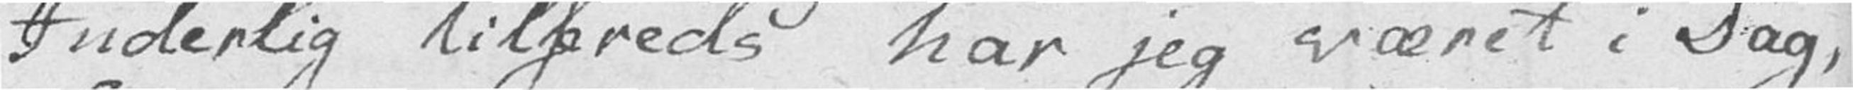Inderlig tilfreds har jeg været i Dag,
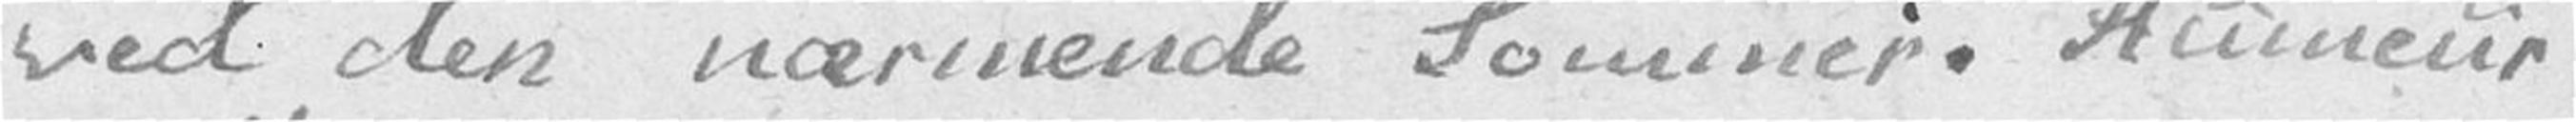ved den nærmende Sommer. Humeur
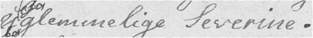uforglemmelige Severine.
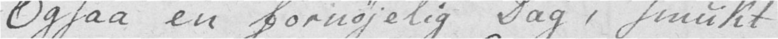Ogsaa en fornøjelig Dag, smukt
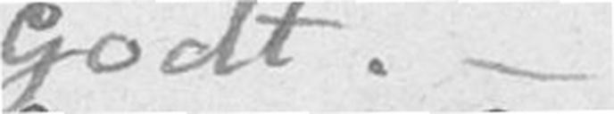Godt. –
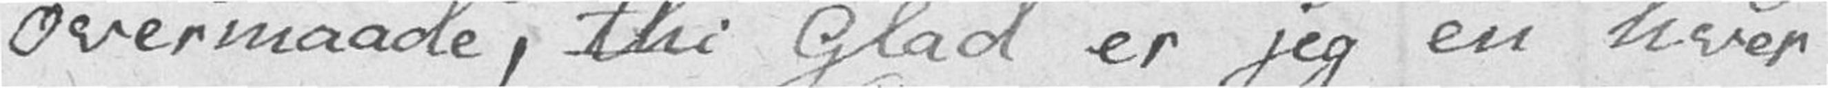overmaade, thi Glad er jeg en hver
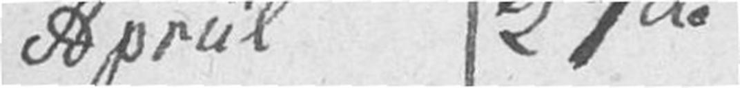Apriil 27de
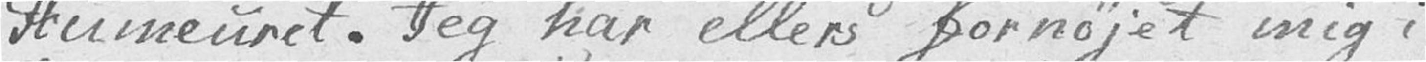Humeuret. Jeg har ellers fornøjet mig i
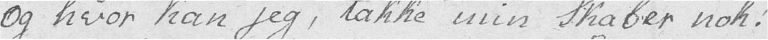og hvor kan jeg, takke min Skaber nok!
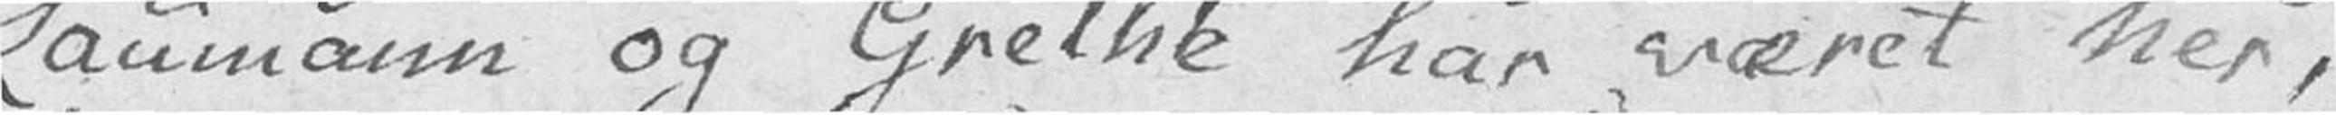Laumann og Grethe har været her,
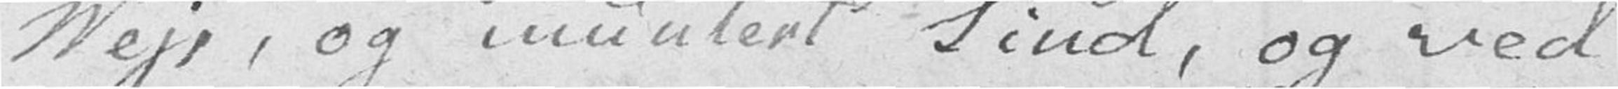Vejr, og muntert Sind, og ved
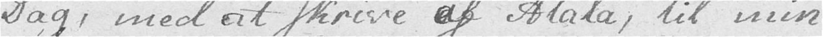Dag, med at skrive af Atala, til min
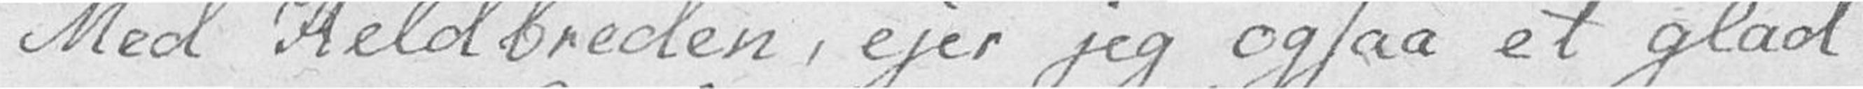Med Heldbreden, ejer jeg ogsaa et glad
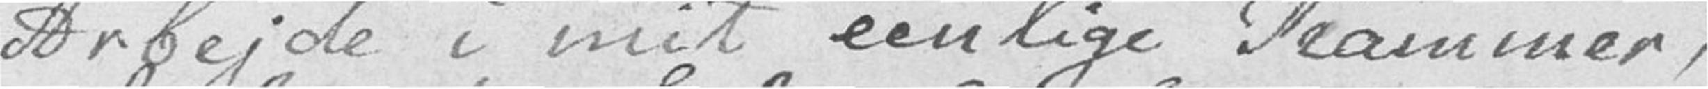Arbejde i mit eenlige Kammer,
